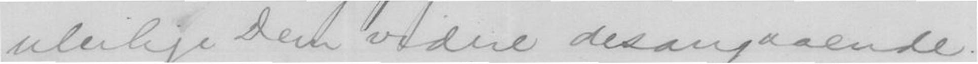uleilige Dem videre desangaaende.
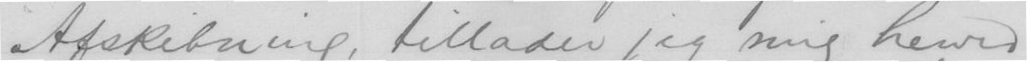Afskibning, tillader jeg mig herved
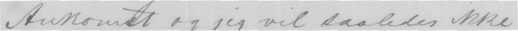Ankomst og jeg vil saaledes ikke
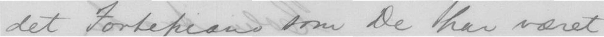det Fortepiano som De har været
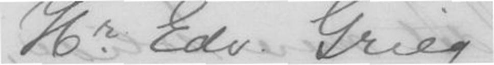Hr Edv. Grieg
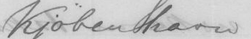Kjøbenhavn
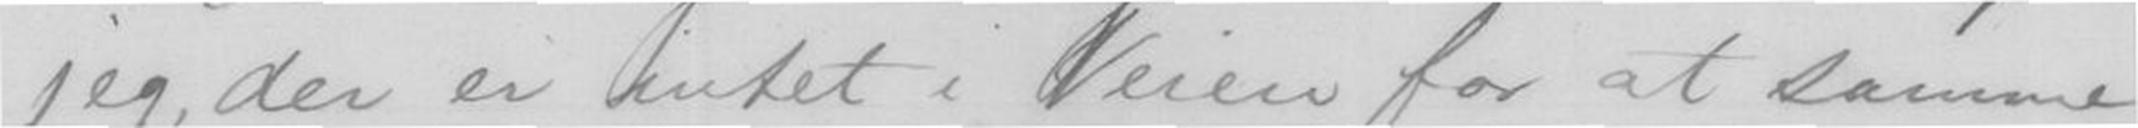jeg, der er intet i Veien for at samme
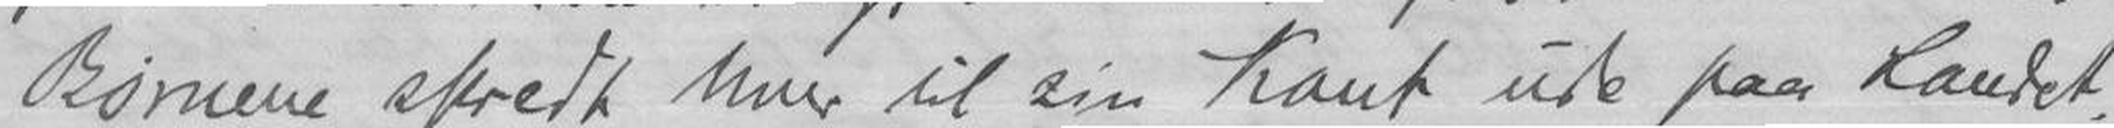Børnene spredt hver til sin Kant ude paa Landet.
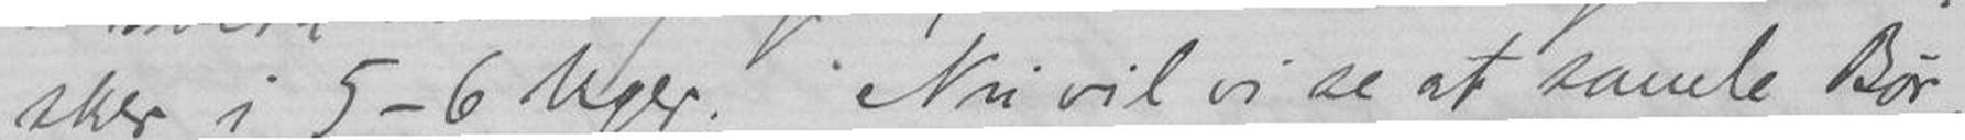sker i 5-6 Uger. Nu vil vi se at samle Bør-
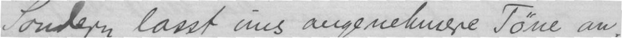"Sondern lasst uns angenehmere Töne an-
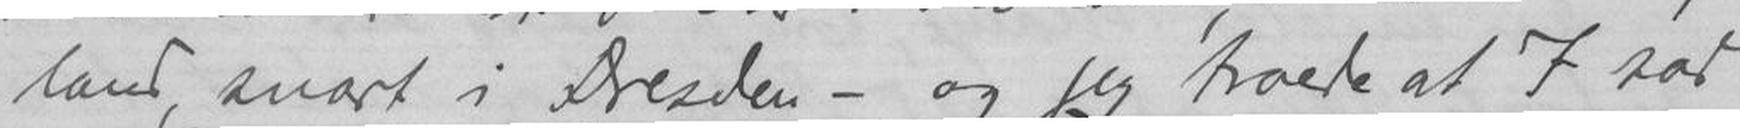land, snart i Dresden - og jeg troede at I sad
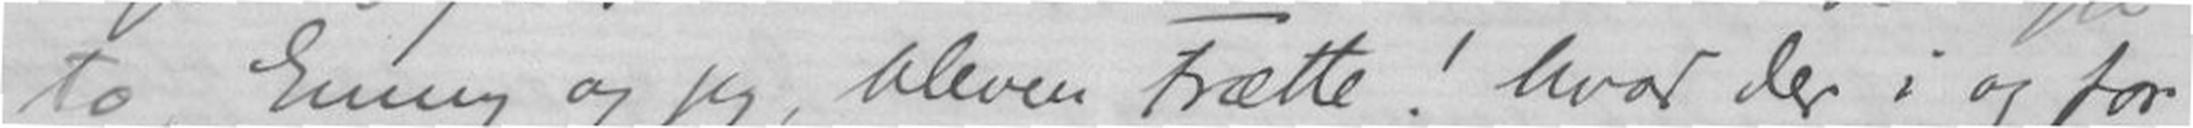to "Emmy og jeg, bleven trætte! hvor der i og for
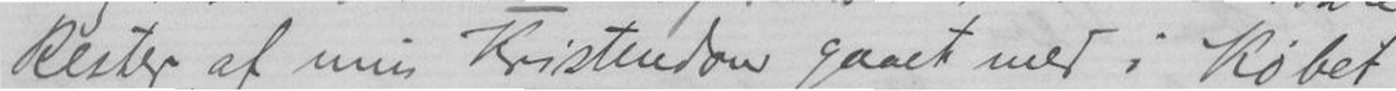Rester af min Kristendom gaaet med i Købet
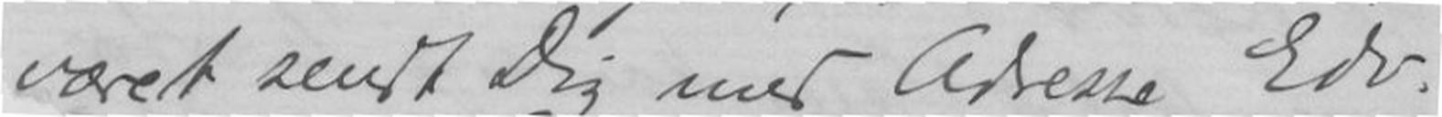været sendt Dig med Adresse Edv.
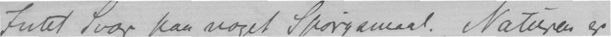Intet svar paa noget Spørgsmaal. Naturen er
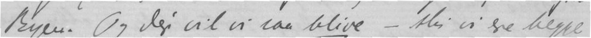Byen. Og deri vil vi saa blive - thi vi ere begge
